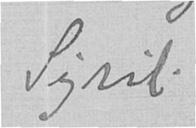Sigrid.
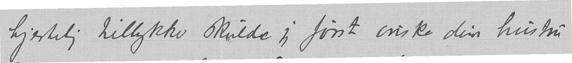hjertelig tillykke skulde jeg først ønske din hustru
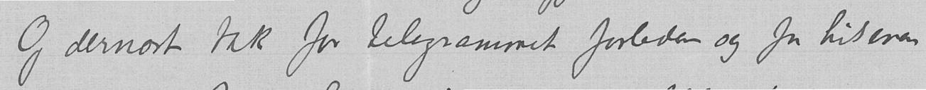Og dernæst tak for telegrammet forleden og for hilsenen
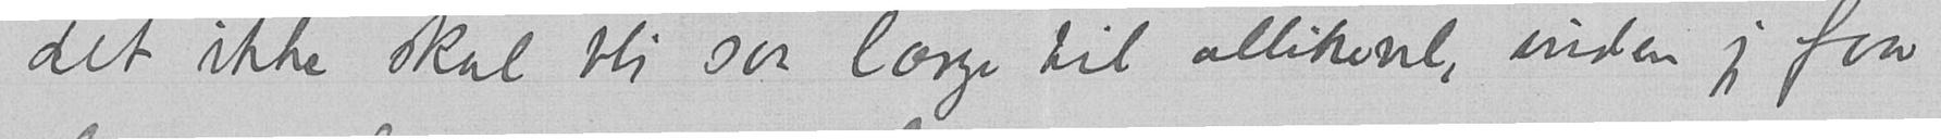det ikke skal bli saa længe til allikevel, inden jeg faar
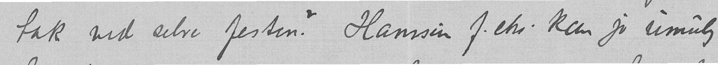tak ved selve festen? Hanssen f.eks. kan jo umulig
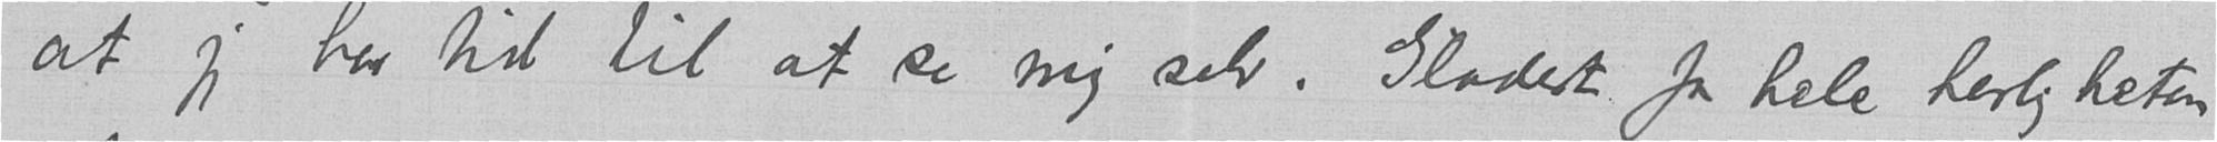at jeg har tid til at se mig selv. Gladest for hele herligheten
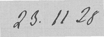23.11.28
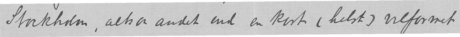Stockholm, altsaa andet end en kort (helst) og velformet
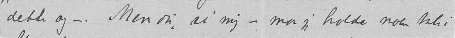dette og –. Men du si mig – maa jeg holde noen tale i
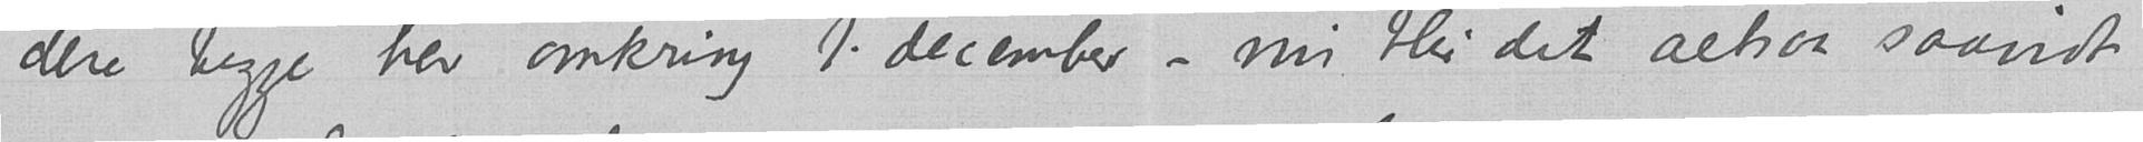dere begge her omkring 1.december – nu blir det altsaa saavidt
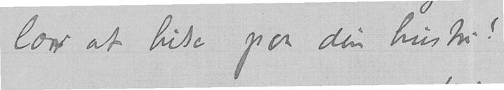lov at hilse paa din hustru!
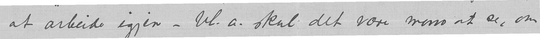at arbeide igjen – bl.a. skal det være morro at se, om
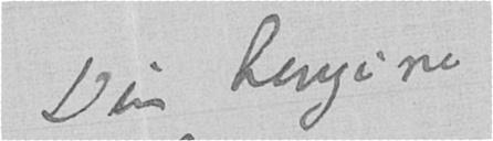Din hengivne
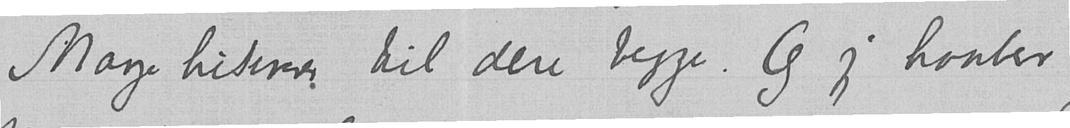Mange hilsener til dere begge. Og jeg haaber
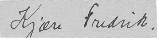Kjære Fredrik,
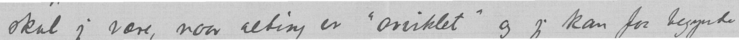skal jeg være, naar alting er "avviklet" og jeg kan faa begynde
